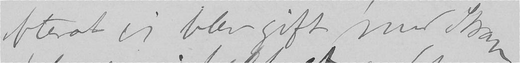efterat jeg blev gift med Skam
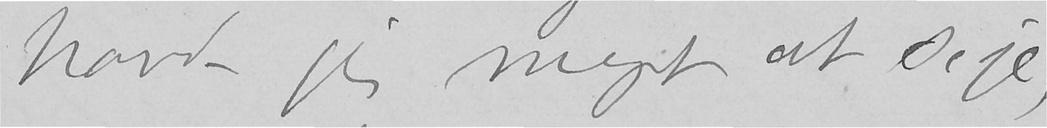havde jeg meget at sige,
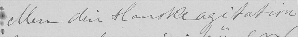Men din Hanskeagitation
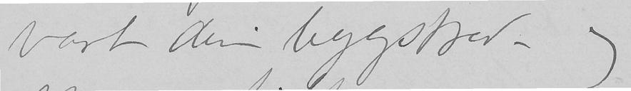vært den begejstrede og
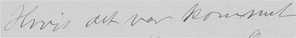Hvis det var kommet
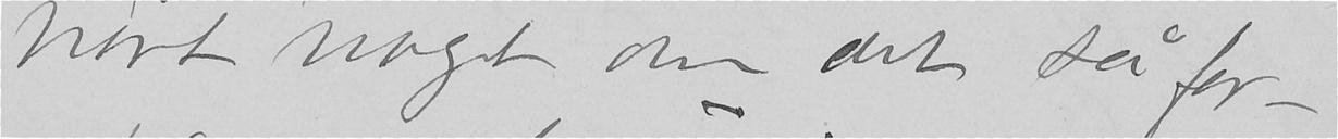hørt noget om det så for-
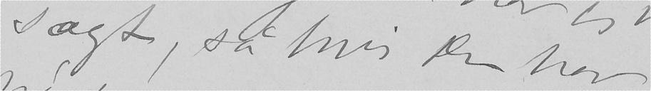sagt, så hvis Du har
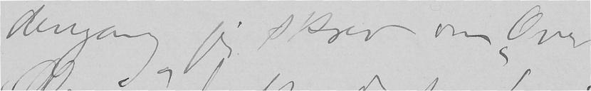dengang jeg skrev om "Over
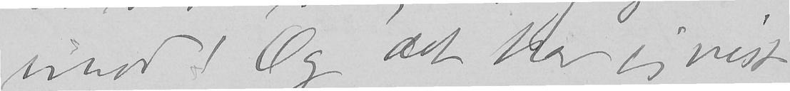imod! Og det har jeg vist
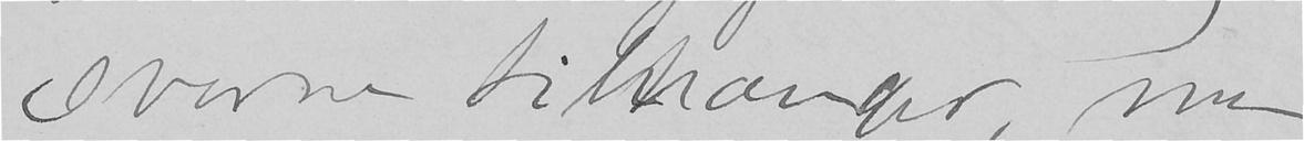svorne tilhænger, men
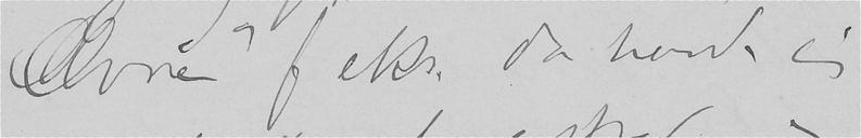Ævne" f eks. da havde jeg
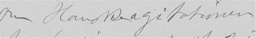om Hanskeagitationen
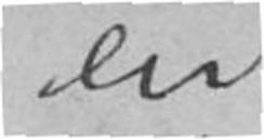en
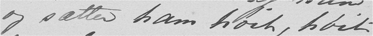og sætter ham høit, hvit
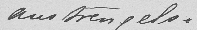anstrengelse.
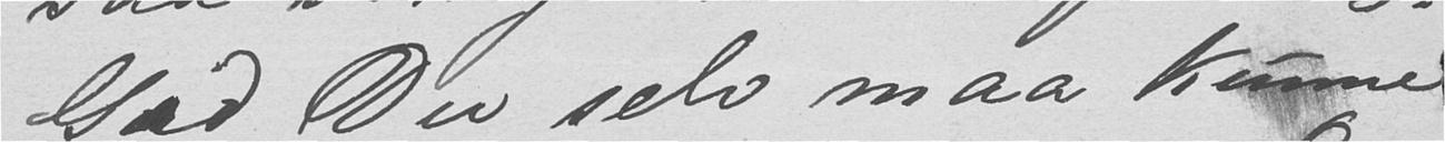Gad Du selv maa kunne
